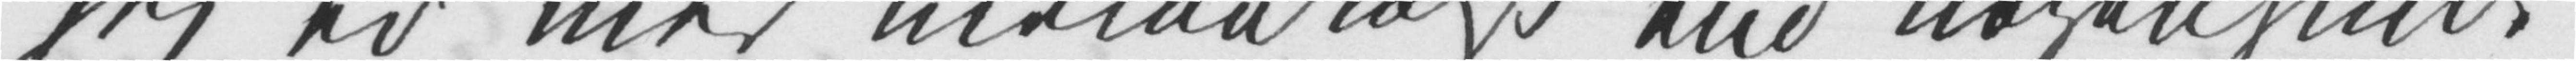jeg er mer melankolsk end nogensinde
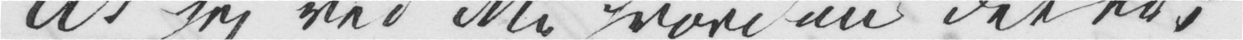Ak jeg ved ikke hvordan det er,
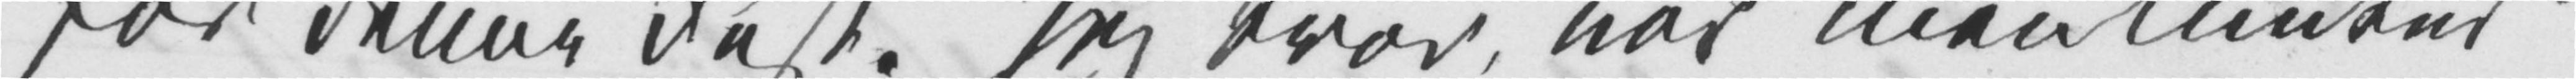før denne Fest. Jeg tror, når man lunker
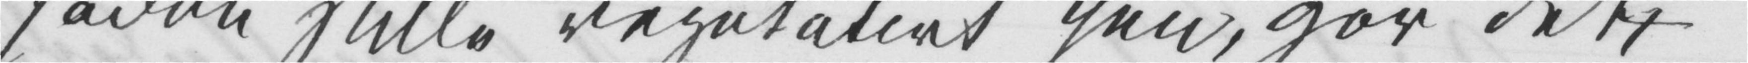sådan stille vegetativt hen, gør det,
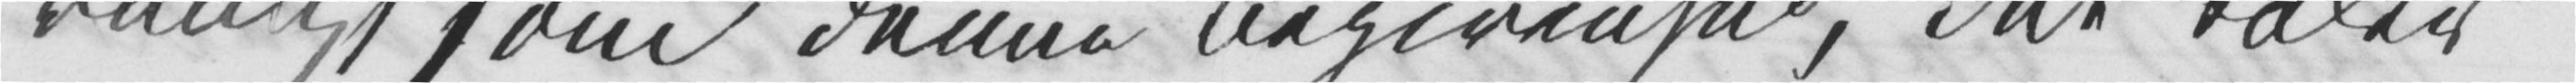vanligt som denne Begivenhed, det tåler
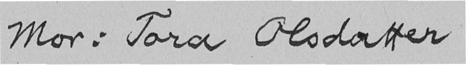Mor: Tora Olsdatter
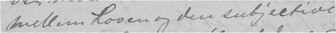mellem Loven og den subjective
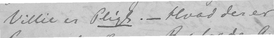Villie er Pligt – Hvad der er
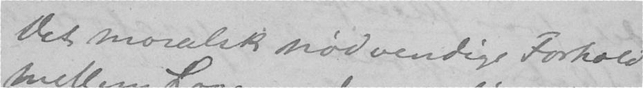Det moralske nødvendige Forhold
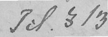Til § 13
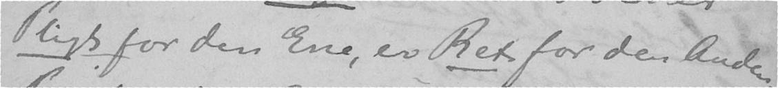Pligt for den Ene, er Ret for den Anden.
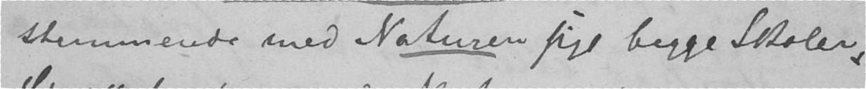stemmende med Naturen sige begge Skoler,
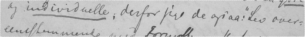og individuelle; derfor sige de ogsaa: "Lev over-
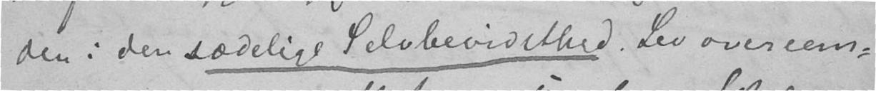den i den sædelige Selvbevidsthed. Lev overeens-
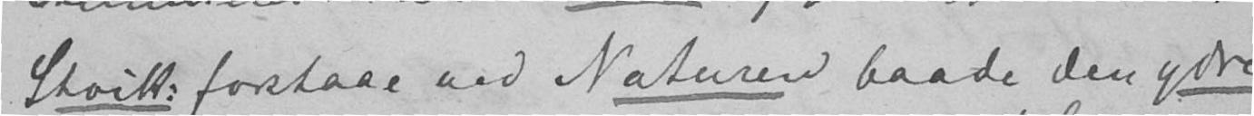Stoik: forstaar ved Naturen baade den ydre
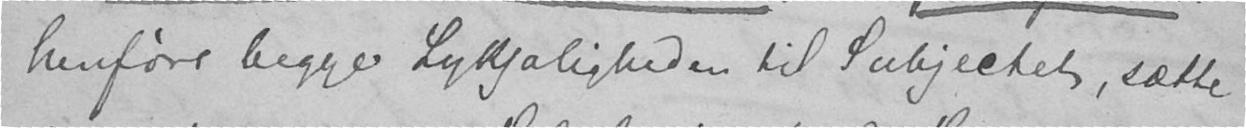henføre begge Lyksaligheden til Subjectet, sætte
